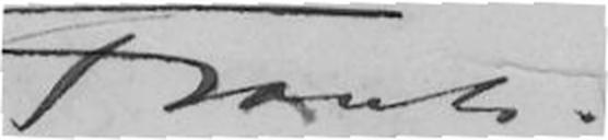Frants.
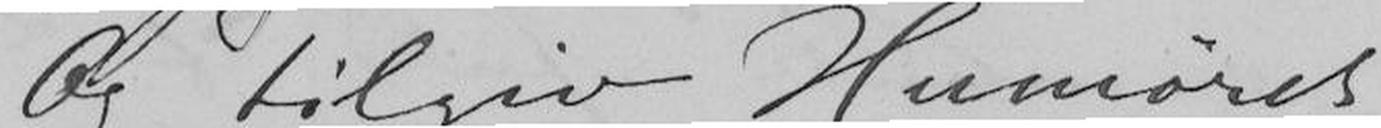Og tilgiv Humøret!
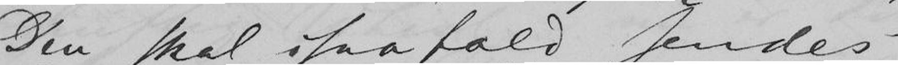Den skal isaafald sendes
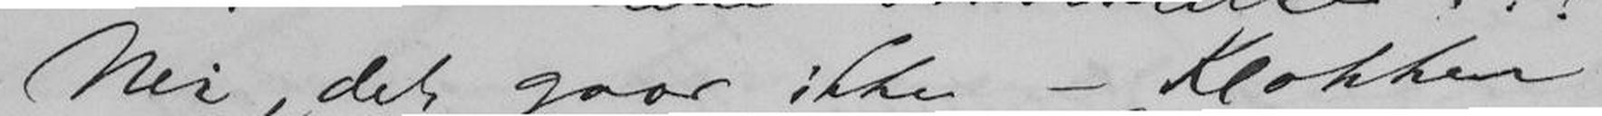Nei, det gaar ikke - Klokken
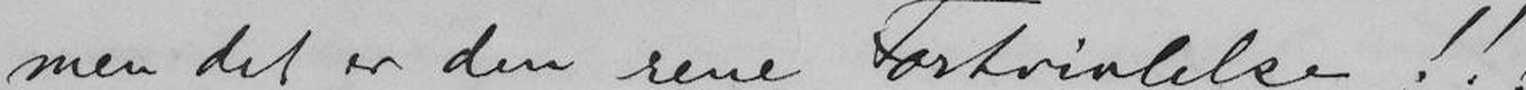men det er den rene Fortvivlelse!!!
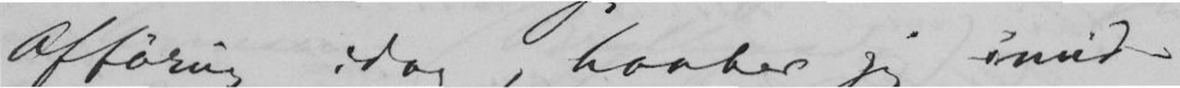Afføring idag, haaber jeg imid-
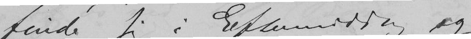finde sig i Eftermiddag og
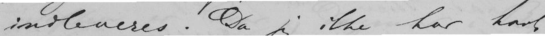indleveres. Da jeg ikke har havt
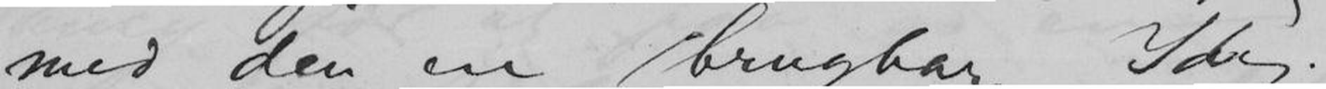med den en brugbar Idé.
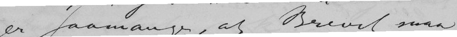er saamange, at Brevet maa
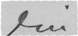Din
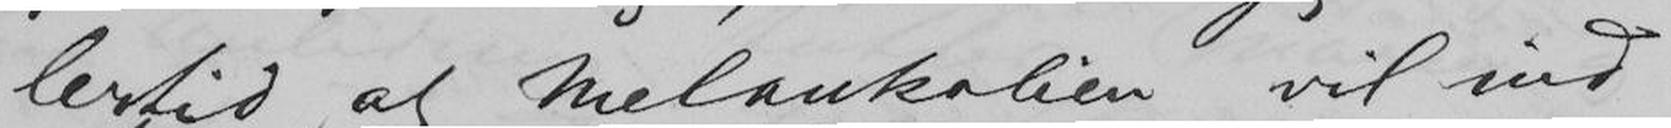lertid, at Melankolien vil ind-
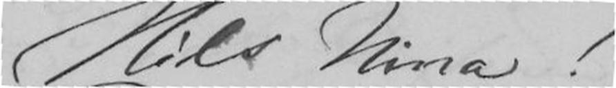Hils Nina!
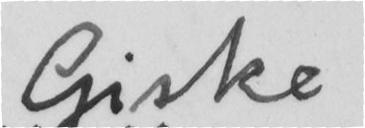Giske
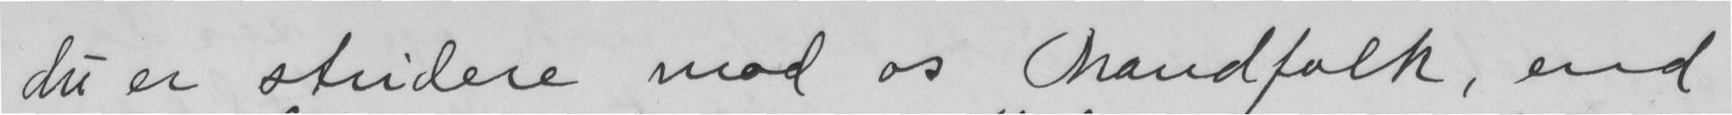du er studere mod os Mandfolk, end
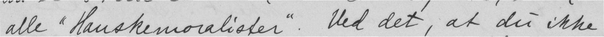alle "Hanskemoralister". Ved det, at du ikke
